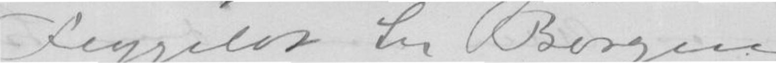Flygelet til Bergen
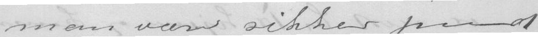man være sikker paa at
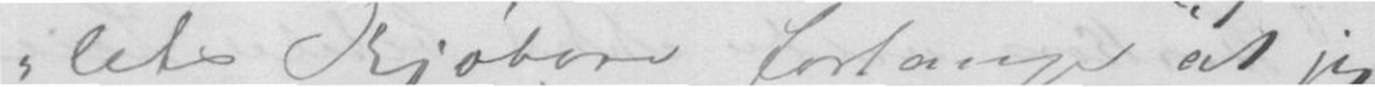lets Kjøbere forlange at jeg
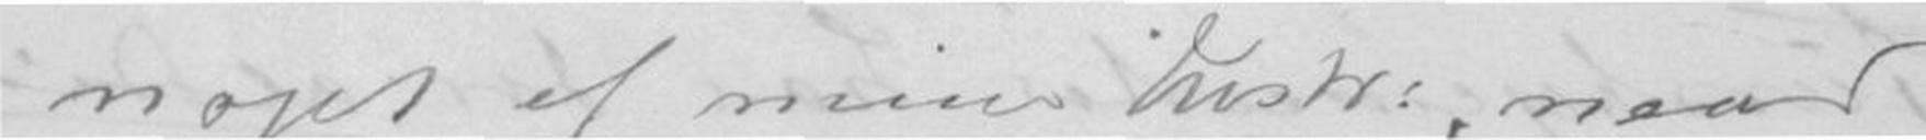noget af mine Instr: naar
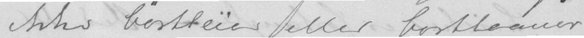ikke bortleier eller bortlaaner
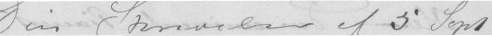Din Skrivelse af 5 Sept
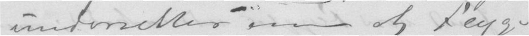underretter om at Flyge-
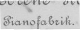Pianofabrik.
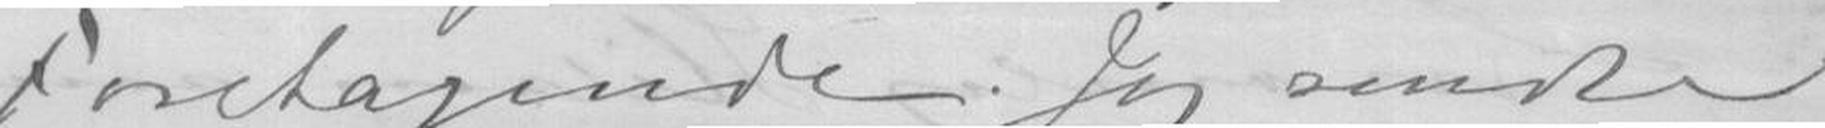Foretagende. Jeg sendte
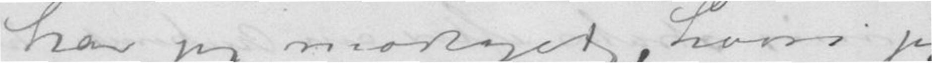har jeg modtaget, hvori jeg
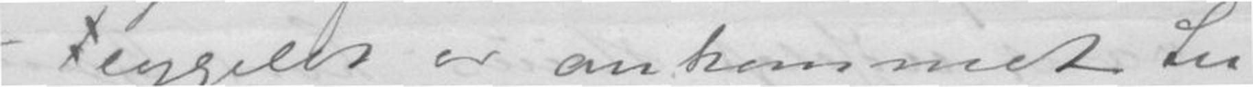Flygelet er ankommet til
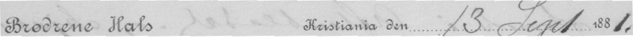Brødrene Hals Kristiania den 13 Sept 1881.
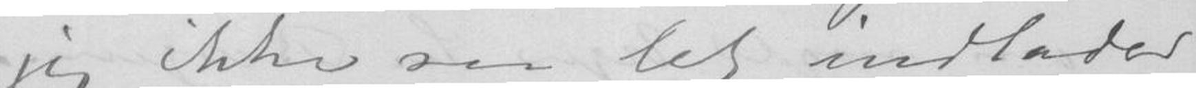jeg ikke saa let indlader
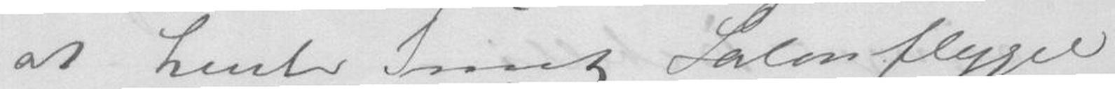at hente mit Salonflygel
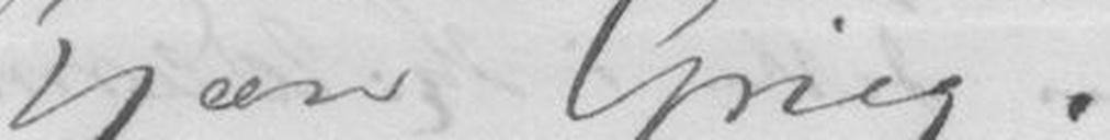Kjære Grieg!
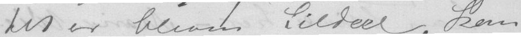det er bleven tildeel, kan
